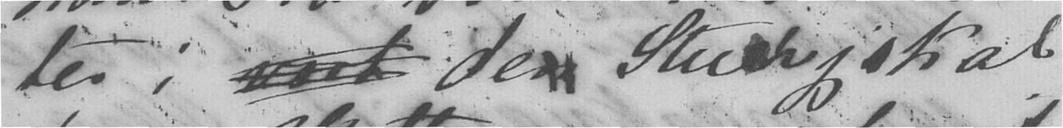ter i vort den Stuer jeg skal
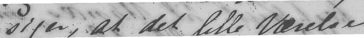siger, at det lille Værelse
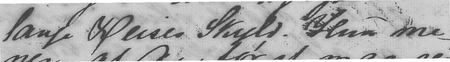lange Reises Skyld. Hun me-
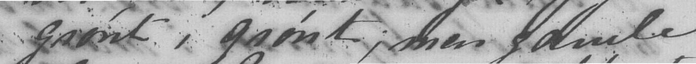grønt i grønt; men gamle
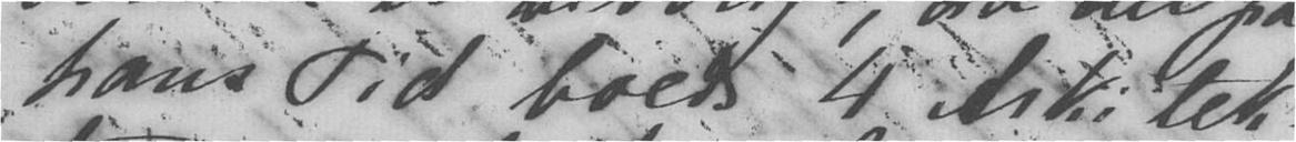hans Tid boet 4 Arkitek-
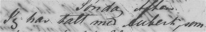Jeg har talt med Aubert, som
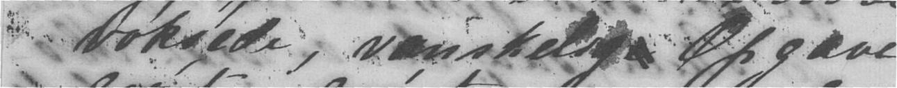voksede, vanskelige Opgave
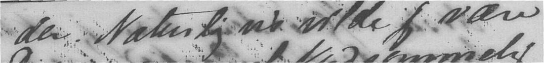der. Naturligvis vilde jeg være
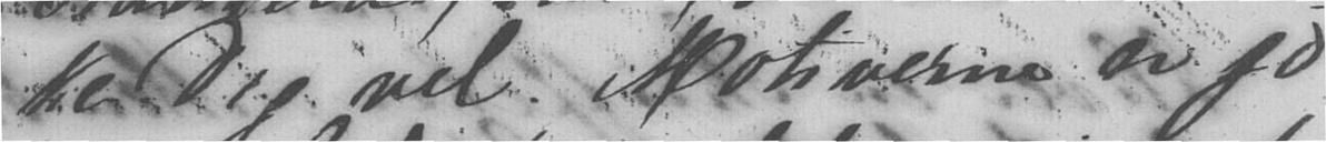ke Dig vel. Motiverne er jo
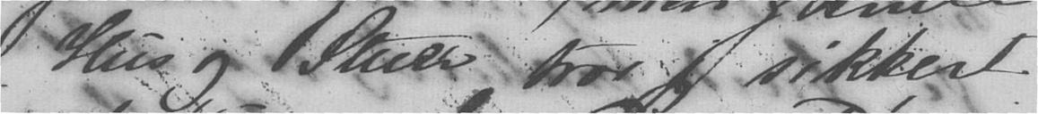Hus og Stuer tror jeg sikkert
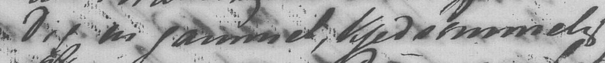Dig en gammel, kjedsommelig
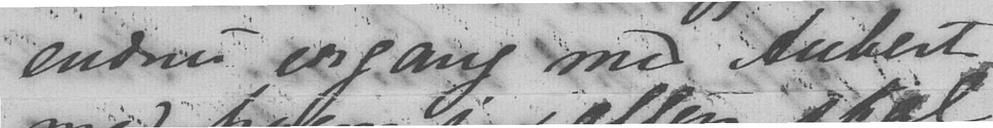endnu engang med Aubert
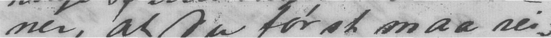ner, at Du først maa rei-
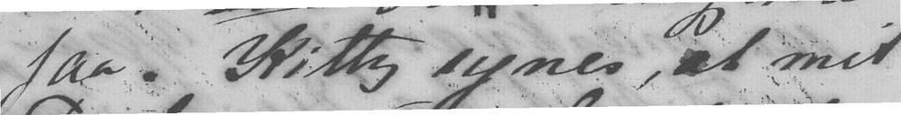faa. Kitty synes, at mit
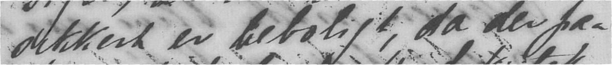sikkert er beboligt, da der paa
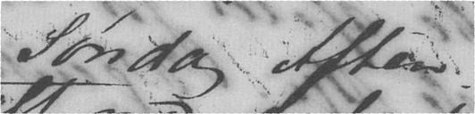Søndag Aften.
